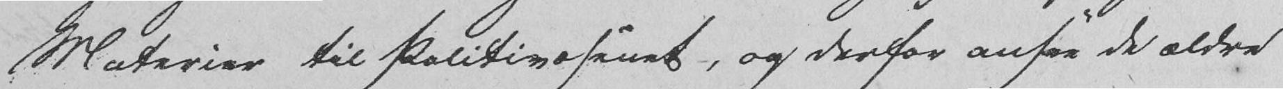Materier til Politivæsenet, og derfor ansee de ældre
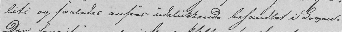liti og saaledes ansees udelukkende behandlet i Loven.
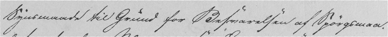Synsmaade til Grund for Besvarelsen af Spørgsmaa-
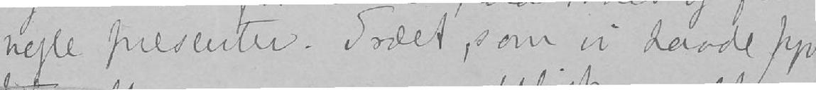nogle presenter. Træet, som vi havde pyn-
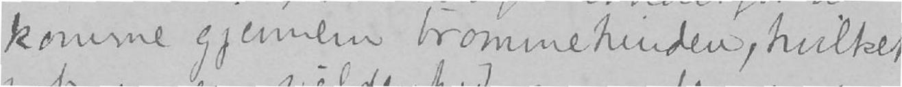komme gjennem trommehinden, hvilket
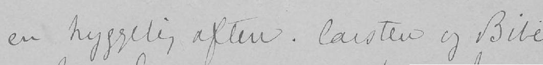en hyggelig aften. Carsten og Bibi
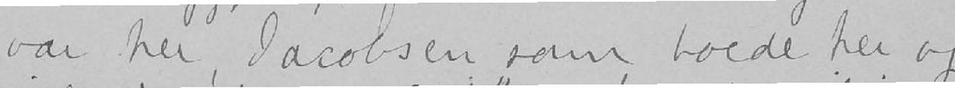var her, Jacobsen som boede her og
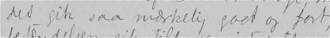det gik saa mærkelig godt og fort,
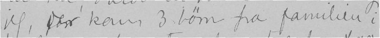jeg, der kom 3 børn fra familien i
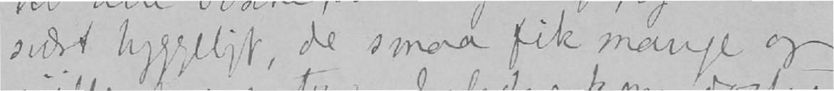svært hyggeligt, de smaa fik mange og
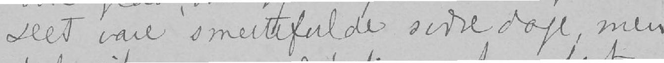Det vare smertefulde svære dage, men
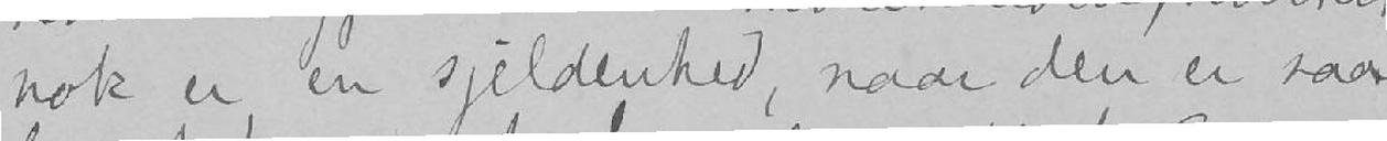nok er en sjeldenhed, naar den er saa
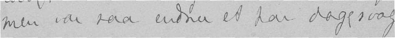men var saa endnu et par dage svag
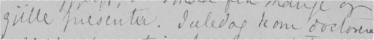gjille presenter. Juledag kom doctoren
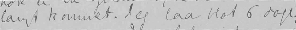langt kommet. Jeg laa blot 6 dage,
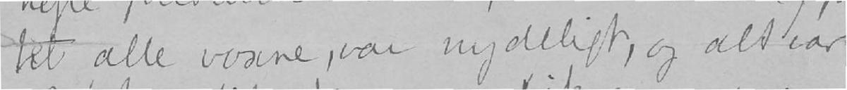tet alle voxne, var nydeligt, og alt var
